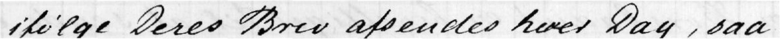ifølge Deres Brev afsendes hver Dag, saa
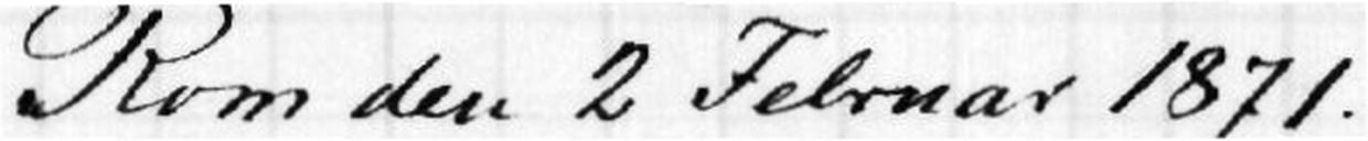Rom den 2 Februar 1871.
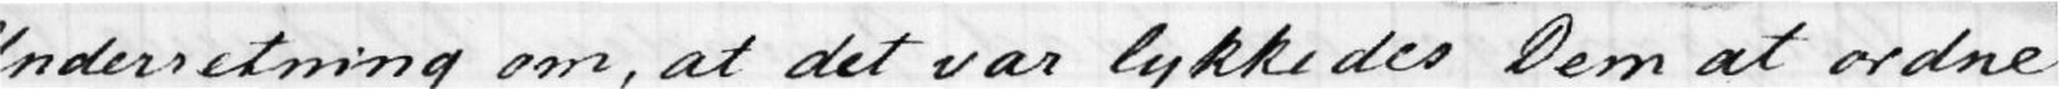med Underretning om, at det var lykkedes Dem at ordne
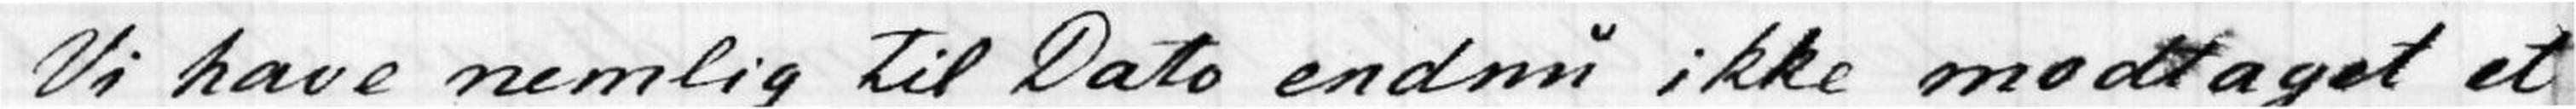Vi have nemlig til Dato endnu ikke modtaget et
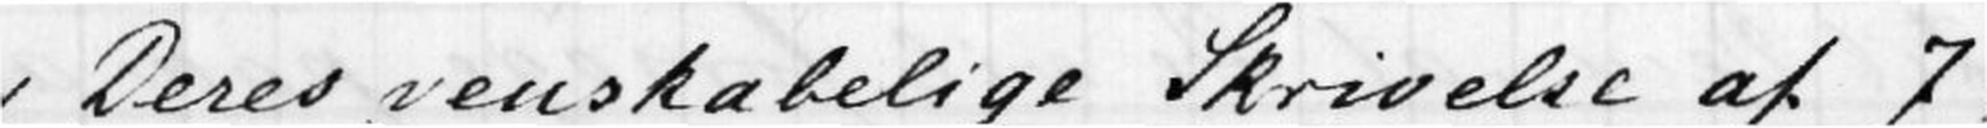for Deres venskabelige Skrivelse af 7
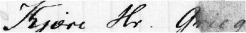Kjære Hr. Grieg!
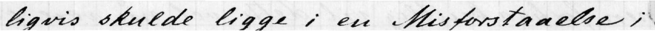ligvis skulde ligge i en Misforstaaelse i
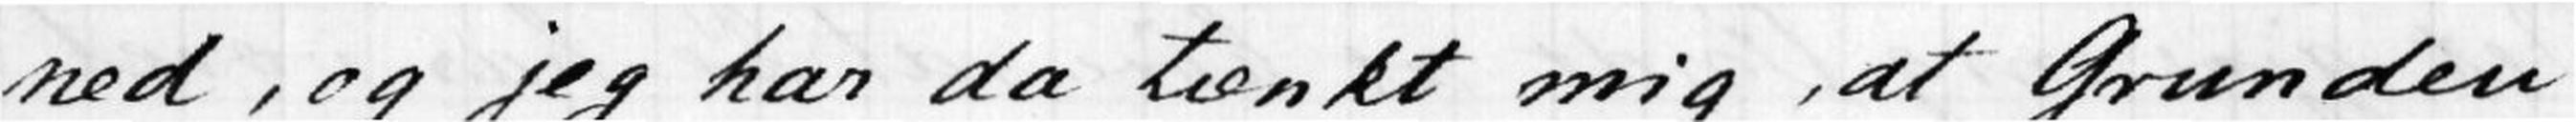ned, og jeg har da tænkt mig at Grunden
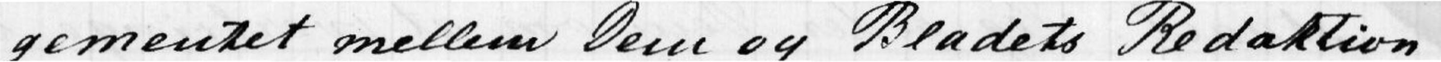gementet mellem Dem og Bladets Redaktion
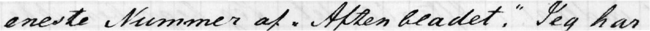eneste Nummer af Aftenbladet. Jeg har
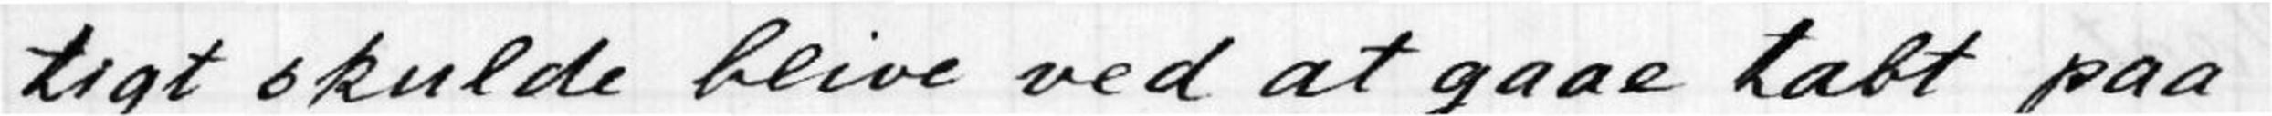tigt skulde blive ved at gaae tabt paa
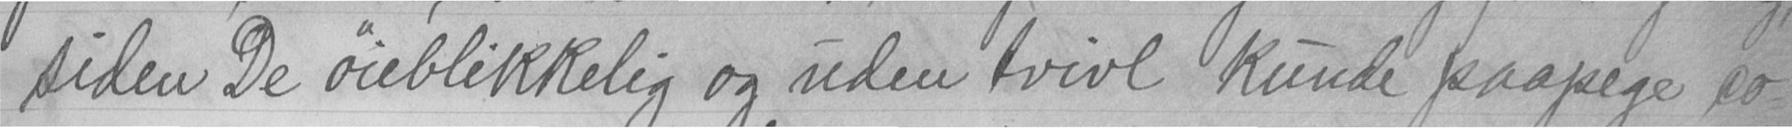siden De øieblikkelig og uden tvivl kunde paapege co-
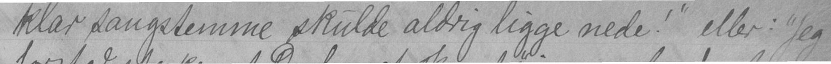klar sangstemme skulde aldrig ligge nede!" eller: "Jeg
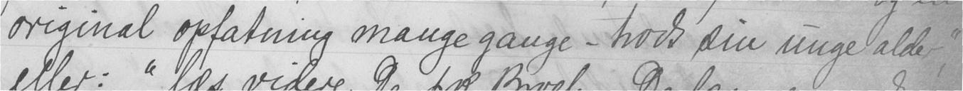original opfatning mange gange - trods sin unge alder,"
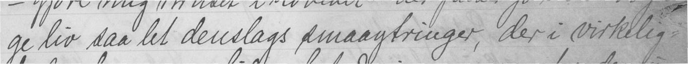ge liv saa let denslags smaaytringer, der i virkelig-
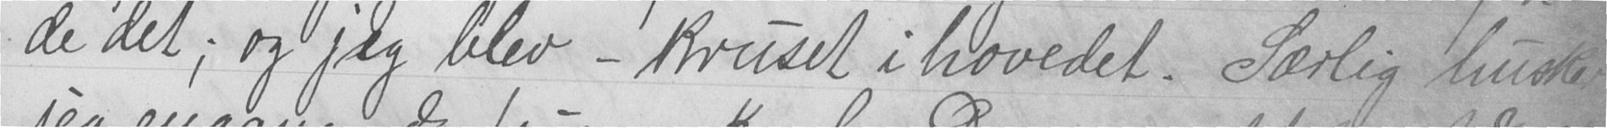de det; og jeg blev - kruset i hovedet. Særlig husker
